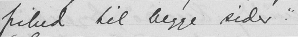frihed til begge sider."
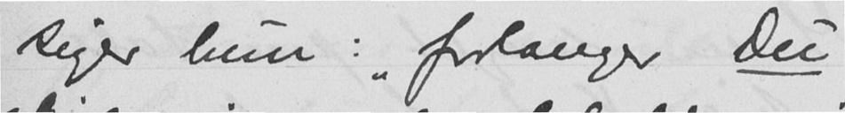siger hun: "forlanger Du
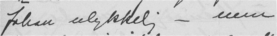Johan ulykkelig - men
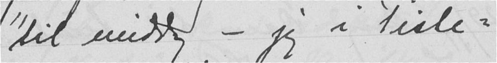til middag - jeg i Tiste-
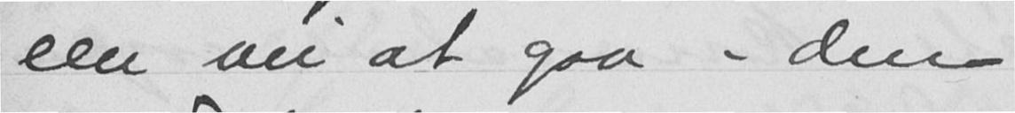een vei at gaa - den
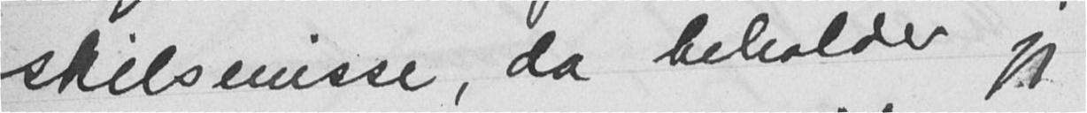skilsmisse, da beholder jeg
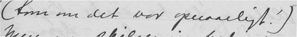(Som om det var opnaaeligt!)
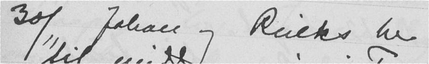30/11 Johan og Riecks her
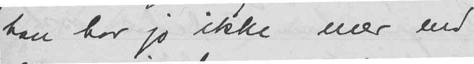han har jo ikke mer end
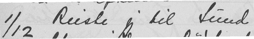1/12 Reiste jeg til Sund
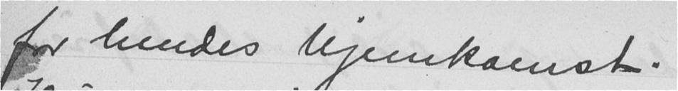for hendes hjemkomst.
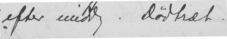efter middag. Dødtræt.
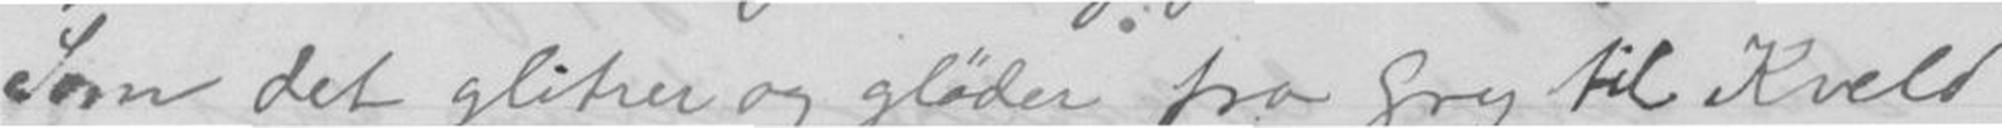Som det glitrer og gløder fra Gry til Kveld
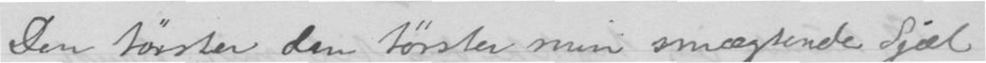Den tørster den tørster min smægtende sjæl
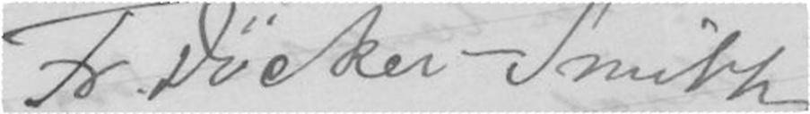Fr. Döcker-Smith
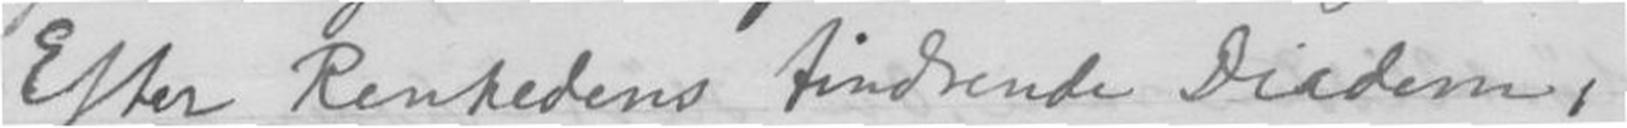Efter Renhedens tindrende Diadem,
